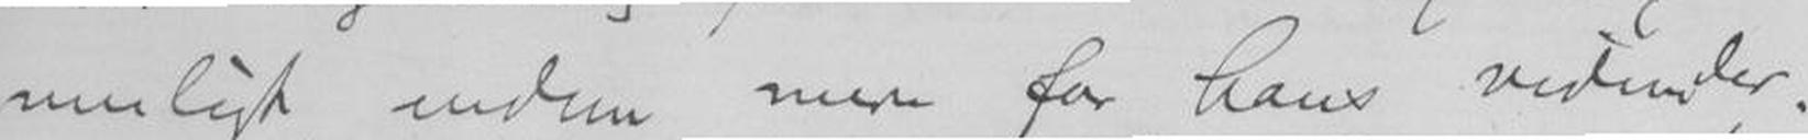muligt endnu mere for hans vidunder-
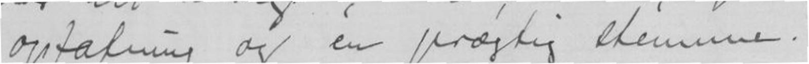opfatning og en prægtig stemme.
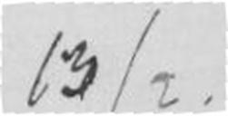13/2.
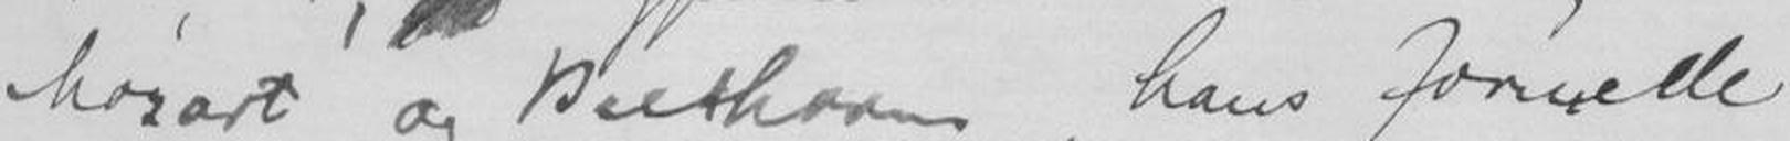Mozart og Bethovens, hans formelle
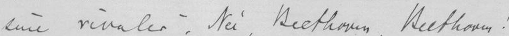sine rivaler". Nei, Beethoven, Beethoven!
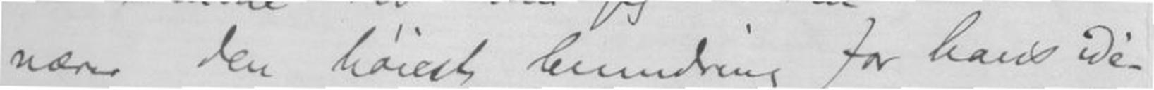nære den høiest beundring for hans idé-
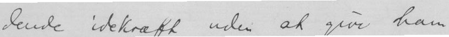dende idekraft uden at give ham
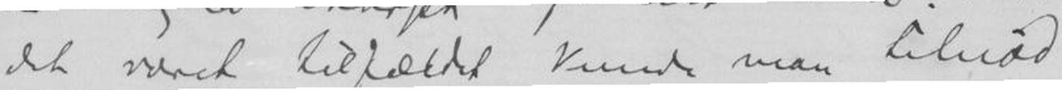det været tilfældet kunde man tilnød
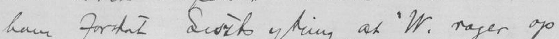have forståt Liszts ytring at "W. rager op
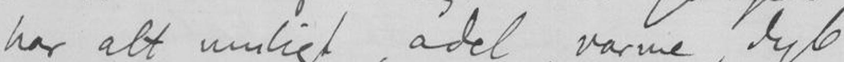har alt venligt, adel, varme, dyb
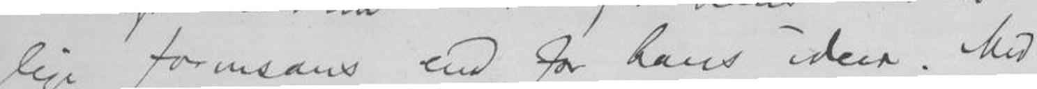lige formsans end for hans ideer. Med
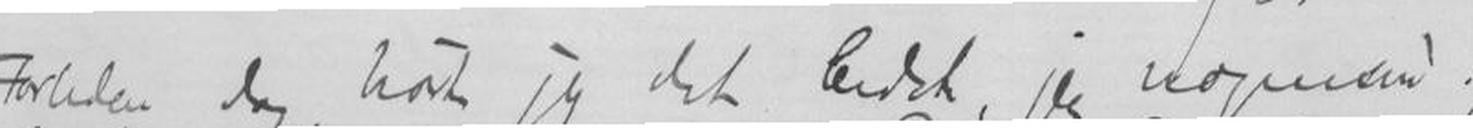Forleden dag hørte jeg det bedste, jeg nogensin-
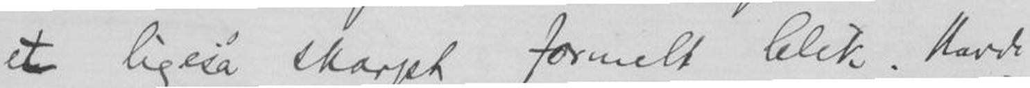et ligeså skarpt formelt blik. Havde
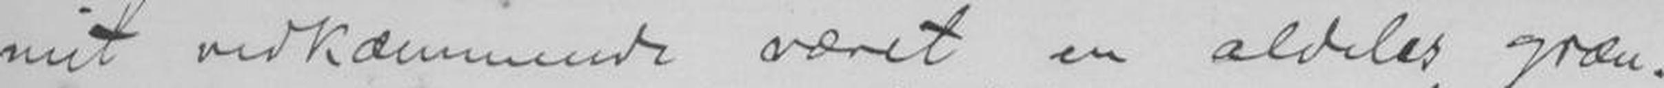mit vedkommende været en aldeles græn-
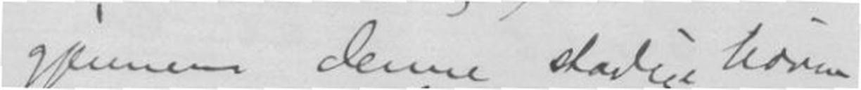gjennem denne stadige høren
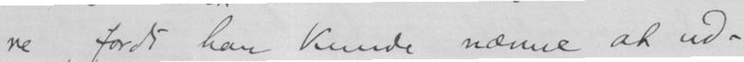re, fordi han kunde nævne at ud-
